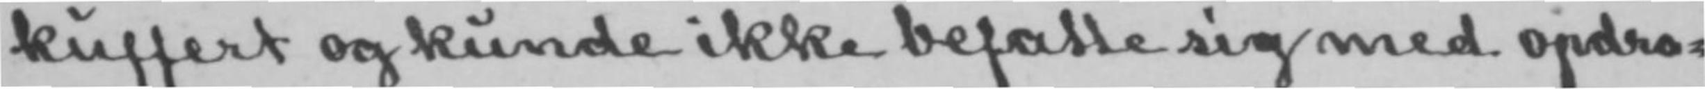kuffert og kunde ikke befatte sig med opdra-
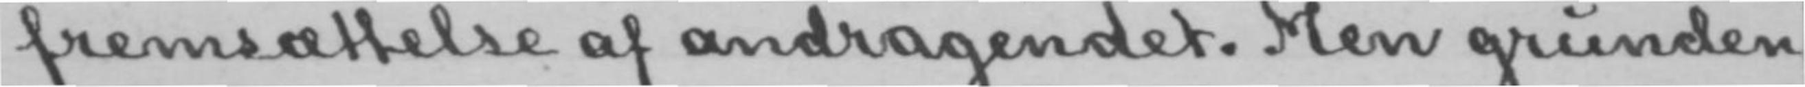fremsættelse af andragendet. Men grunden
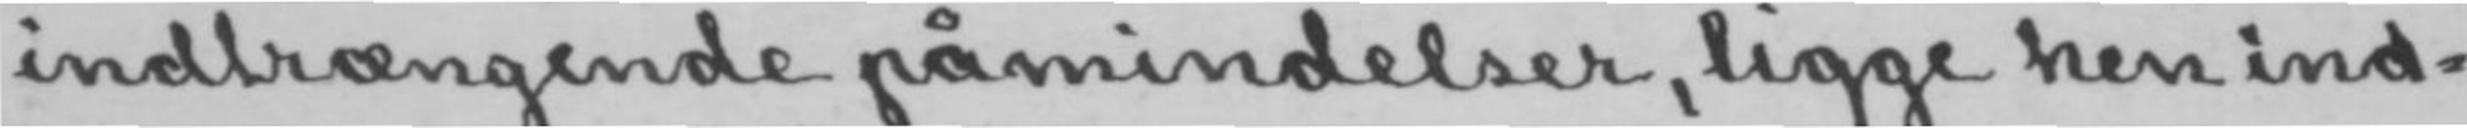indtrængende påmindelser, ligge hen ind-
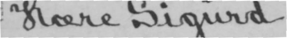Kære Sigurd,
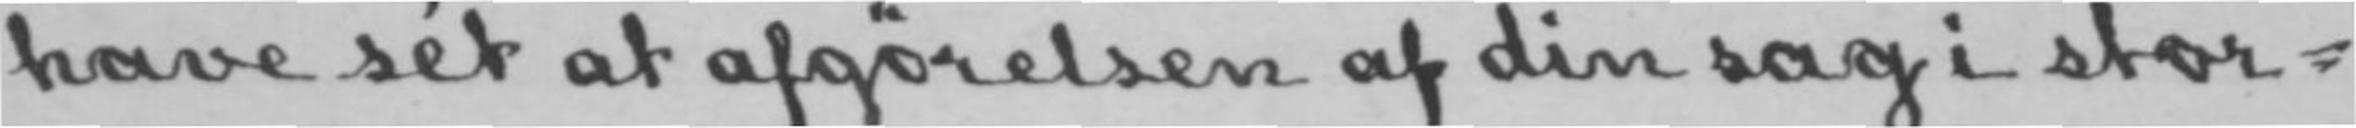have sét at afgørelsen af din sag i stor-
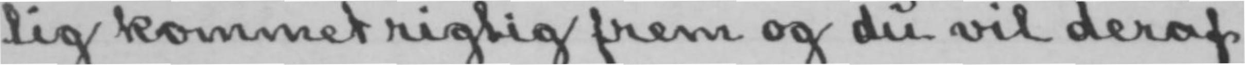lig kommet rigtig frem og du vil deraf
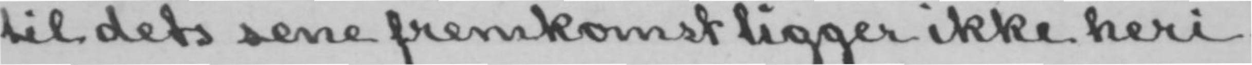til dets sene fremkomst ligger ikke heri.
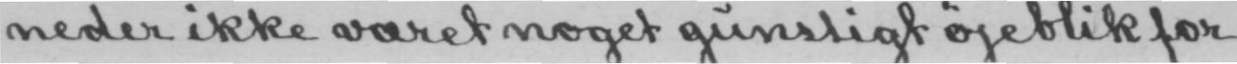neder ikke været noget gunstigt øjeblik for
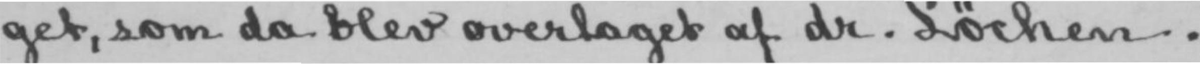get, som da blev overtaget af dr. Løchen.
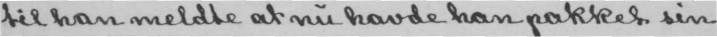til han meldte at nu havde han pakket sin
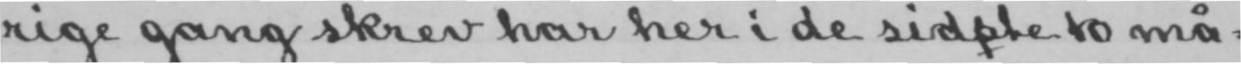rige gang skrev har her i de sidste to må-
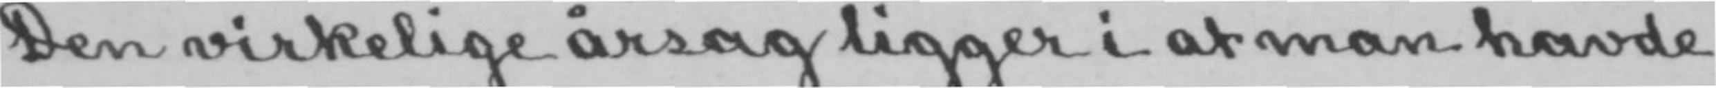Den virkelige årsag ligger i at man havde
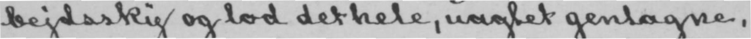bejdssky og lod det hele, uagtet gentagne,
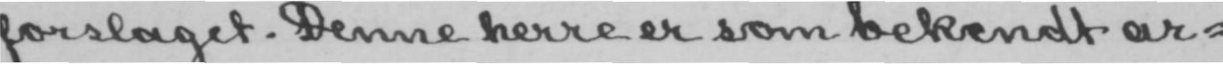forslaget. Denne herre er som bekendt ar-
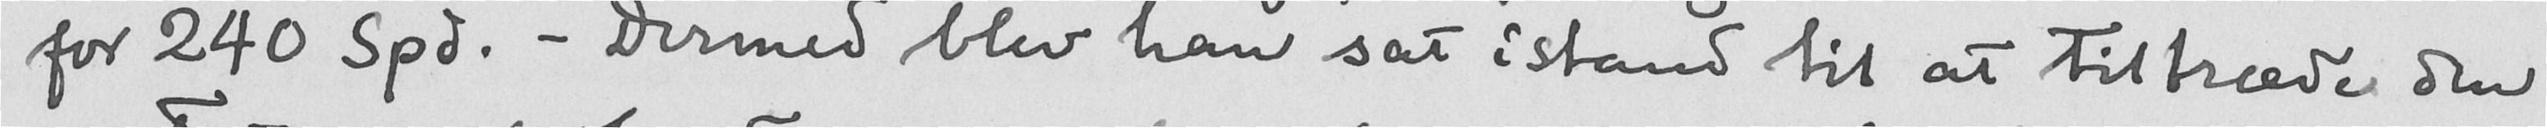for 240 Spd. - Dermed blev han sat istand til at tiltræde den
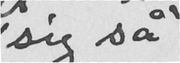sig så
